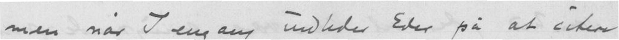men når I engang indleder Eder på at citere
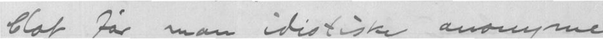blot får men idiotiske anonyme
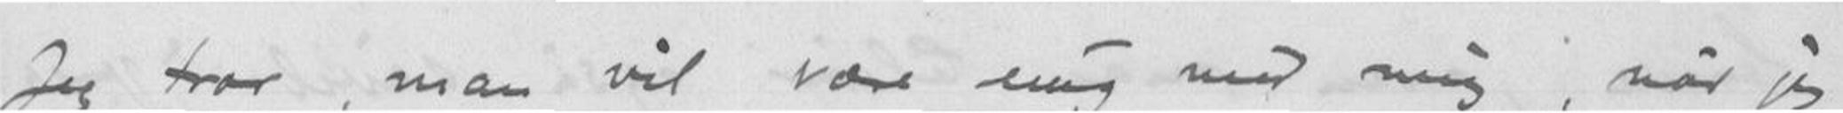Jeg tror, man vil være enig med mig, når jeg
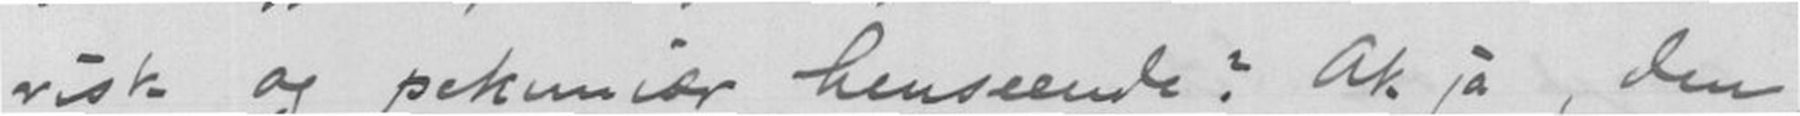risk og pekuniær henseende? Ak ja, den,
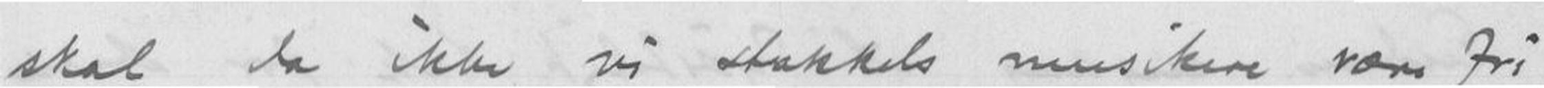skal da ikke vi stakkels musikere være fri
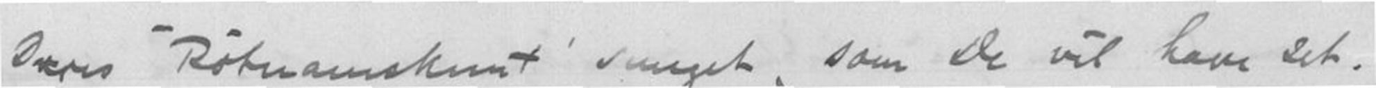Deres "Røtnamskunt" sunget, som De vil have set.
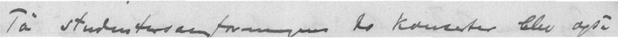På studentersangforreningens to konserter blev også
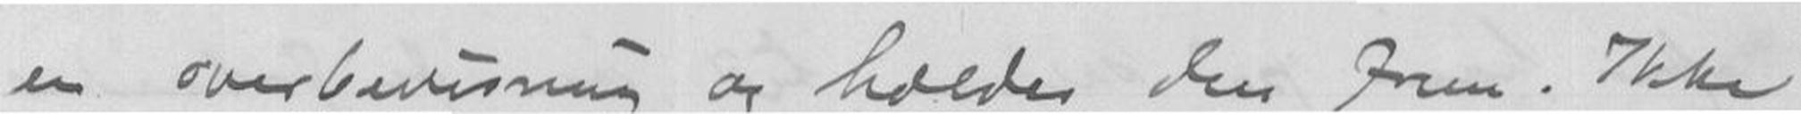en overbevisning og holder den frem. Ikke
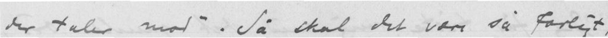der taler mod". Så skal det være så farligt,
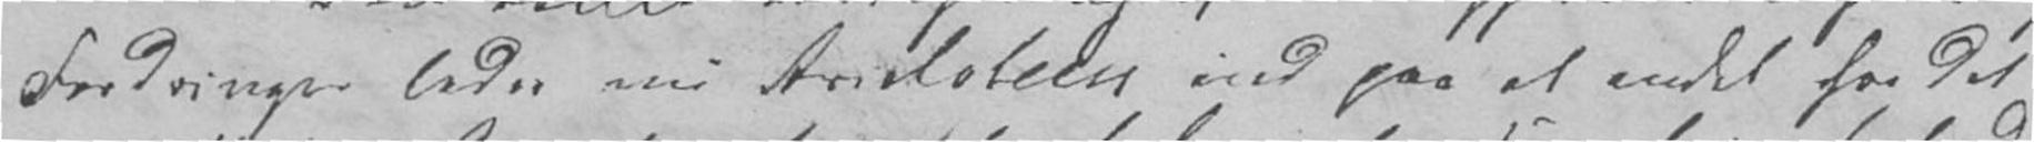Fordringer leder nu Aristoteles ind paa et andet for det
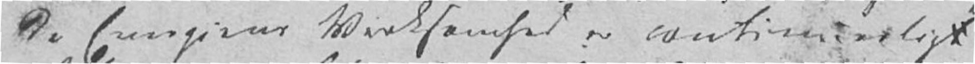da Energiens Virksomhed er continuerligt
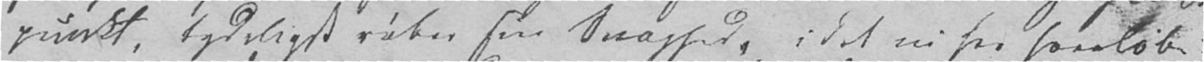punkt, tydeligt røber sin Svaghed, i det vi her foreløbig
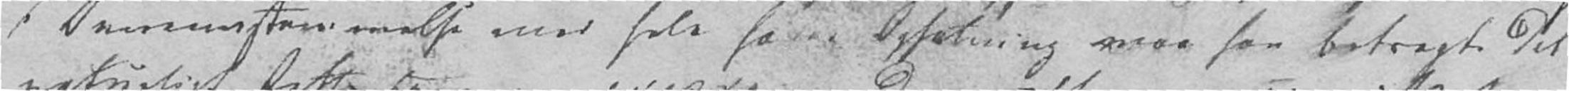i Overeensstemmelse med hele hans Opfatning maa han betragte det
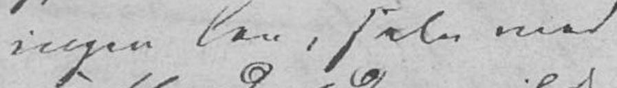ingen Lov, selv med
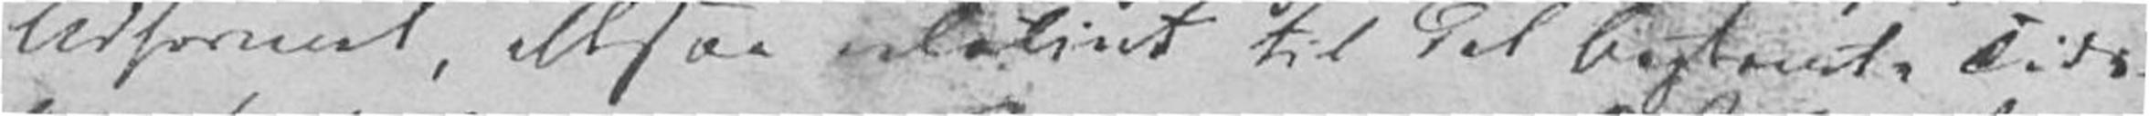Udformet, altsaa relativt til det bestemte Tids-
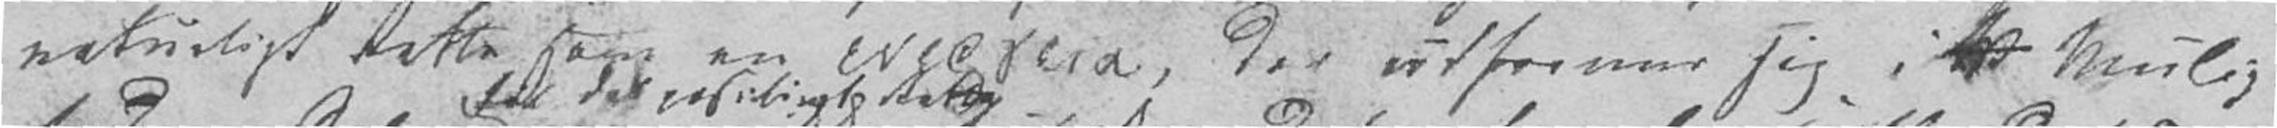naturligt Rette som en gresk, der udformer sig i V Mulig-
